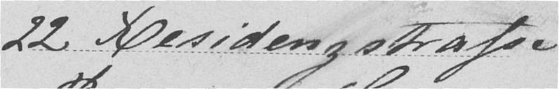22 Residenzstrasse
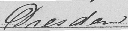Dresden
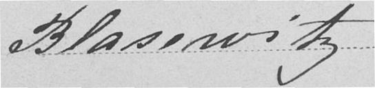Blasewitz
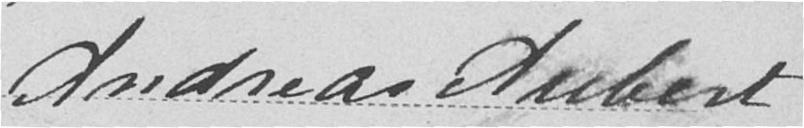Dr. Andreas Aubert
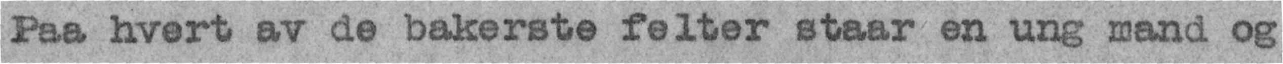Paa hvert av de bakerste felter staar en ung mand og
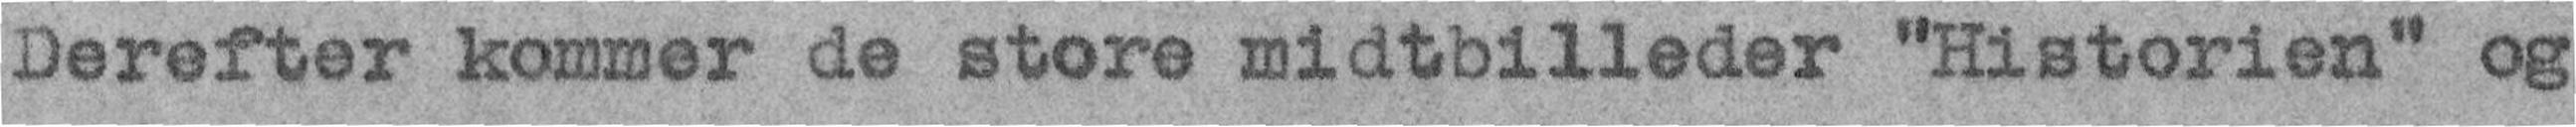derefter kommer de store midtbilleder «Historien» og
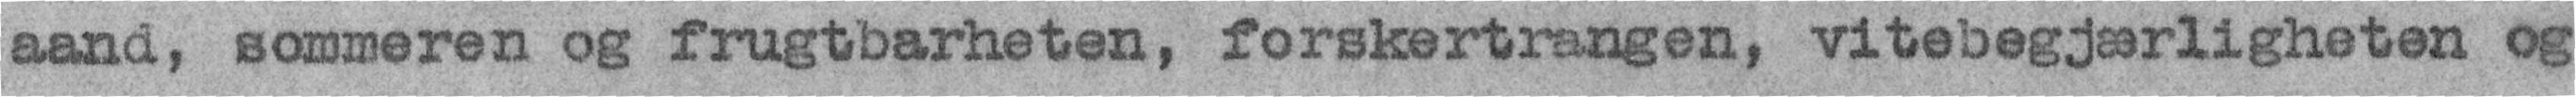aand, sommeren og frugtbarheten, forskertrangen, vitebegjærligheten og
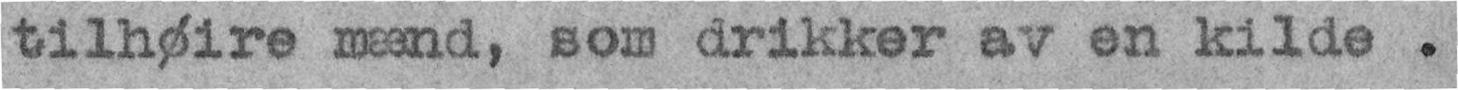tilhøire mænd, som drikker av en kilde.
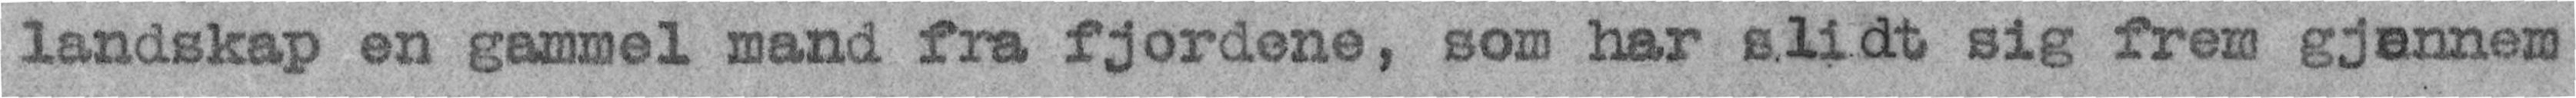landskap en gammel mand fra fjordene, som har slidt sig frem gjennem
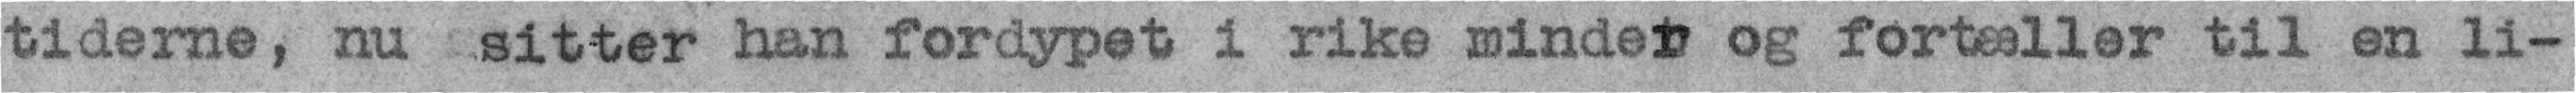tiderne, nu sitter han fordypet i rike minder og fortæller til en li-
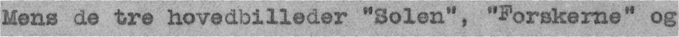Mens de tre hovedbilleder «Solen», «Forskerne» og
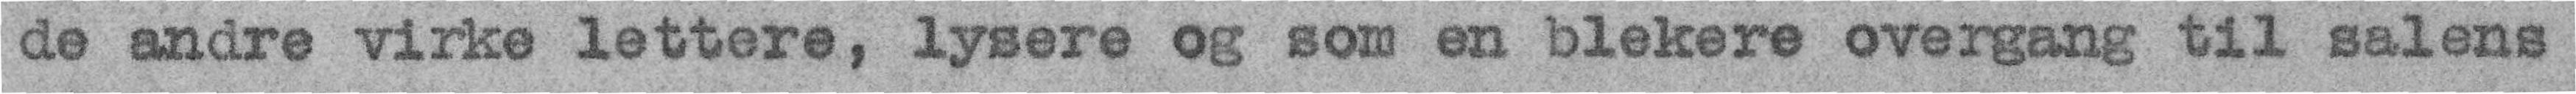de andre virke lettere, lysere og som en blekere overgang til salens
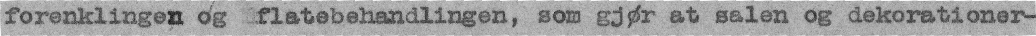forenklingen og flatebehandlingen, som gjør at salen og dekorationer-
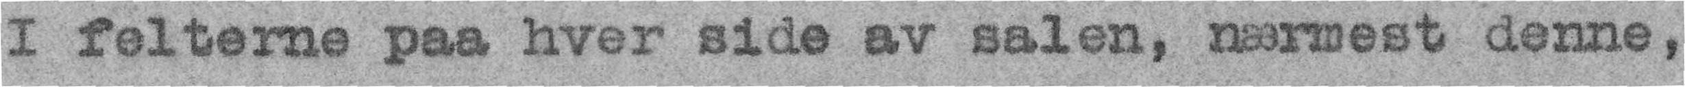I felterne paa hver side av salen, nærmest denne,
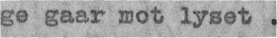ge gaar mot lyset.
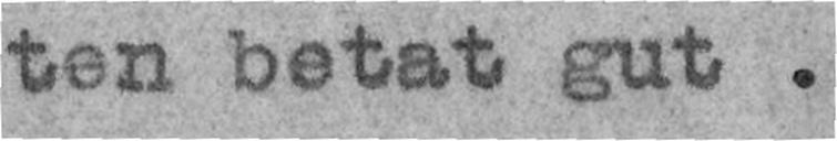ten betat gut.
